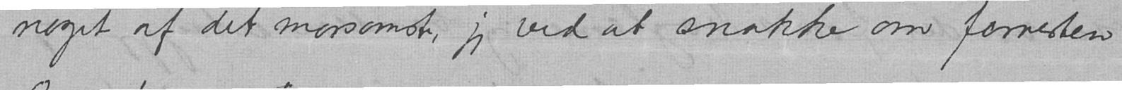noget af det morsomste, jeg ved at snakke om forresten.
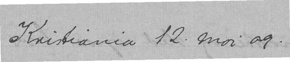Kristiania 12. mai 09.
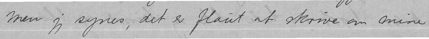Men jeg synes, det er flaut at skrive om mine
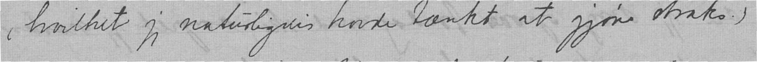(hvilket jeg naturligvis havde tænkt at gjøre straks.)
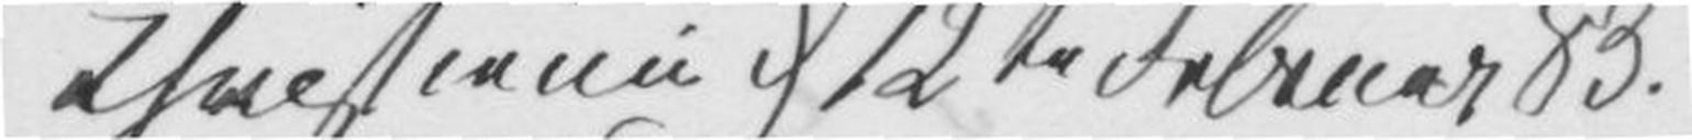Christiania d 12te Februar 83.
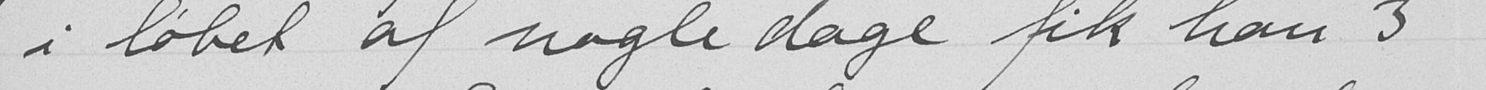i løbet af nogle dage fik han 3
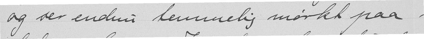og ser endnu temmelig mørkt paa
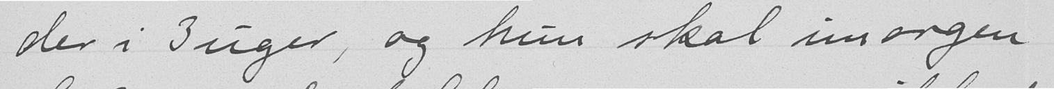der i 3 uger, og hun skal imorgen
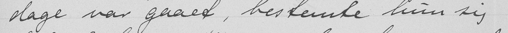dage var gaaet, bestemte hun sig
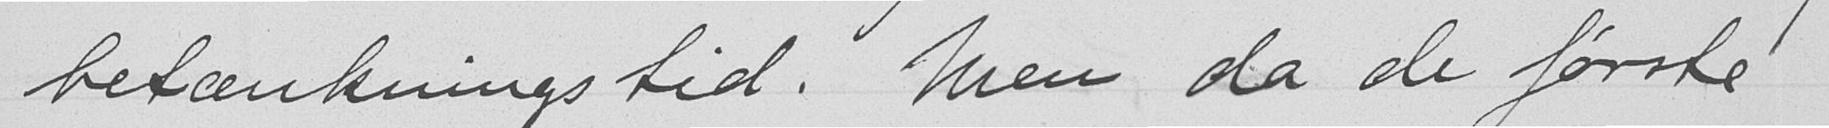betænkningstid. Men da de første
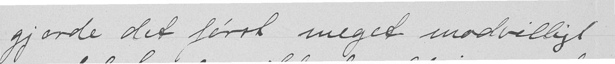gjorde det først meget modvilligt
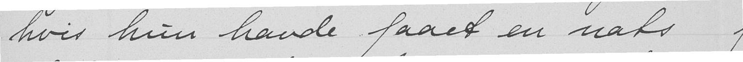hvis hun havde faaet en nats
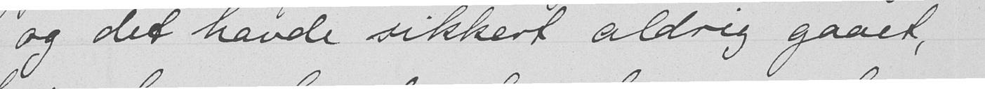- og det havde sikkert aldrig gaaet,
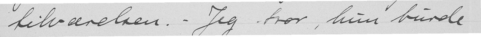tilværelsen. - Jeg tror, hun burde
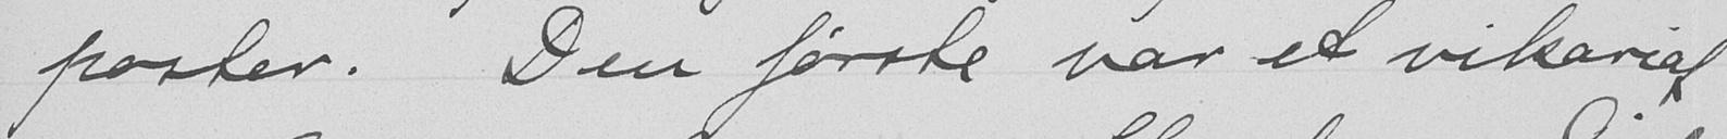poster. Den første var et vikariat
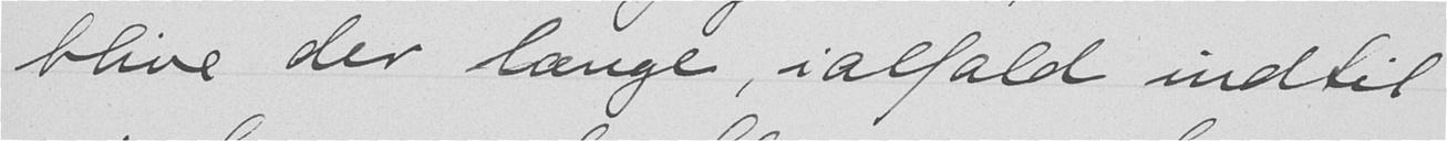blive der længe, ialfald indtil
